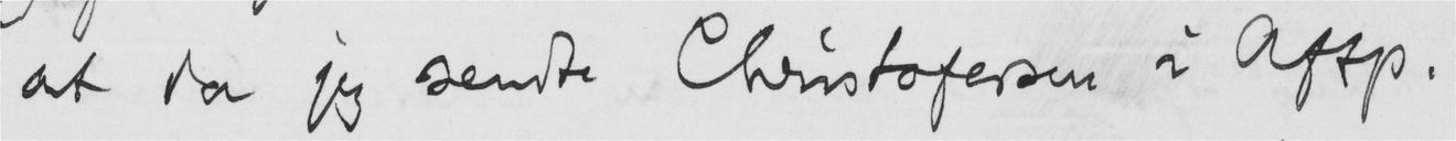at da jeg sendte Christofersen i Aftp.
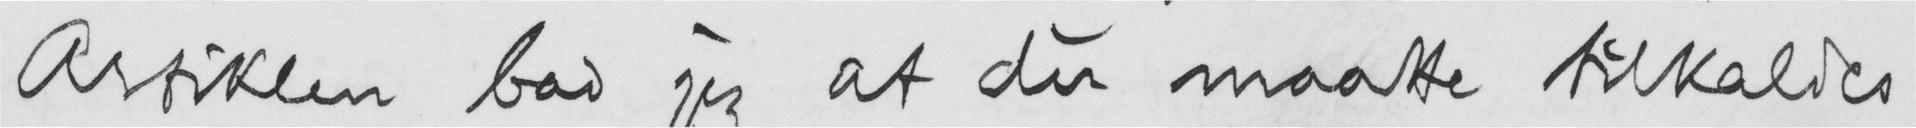Artiklen bad jeg at du maatte tilkaldes
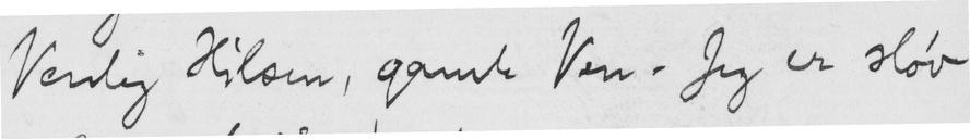Venlig Hilsen, gamle Ven. Jeg er sløv
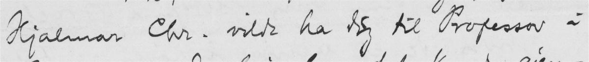Hjalmar Chr. vilde ha dig til Professor i
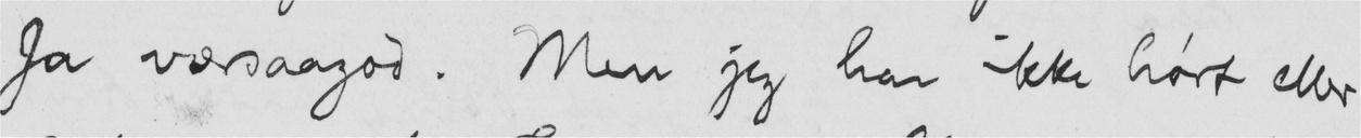Ja værsaagod. Men jeg har ikke hørt eller
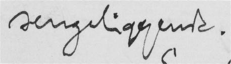sengeliggende.
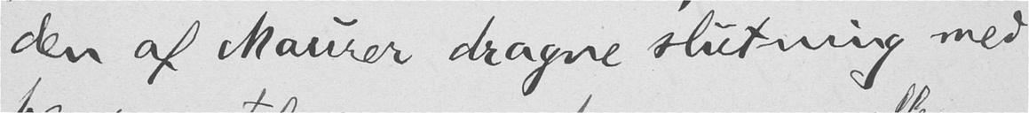den af Maurer dragne slutning med
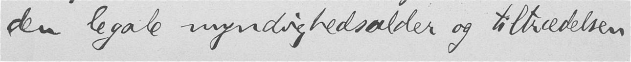den legale myndighedsalder og tiltrædelsen
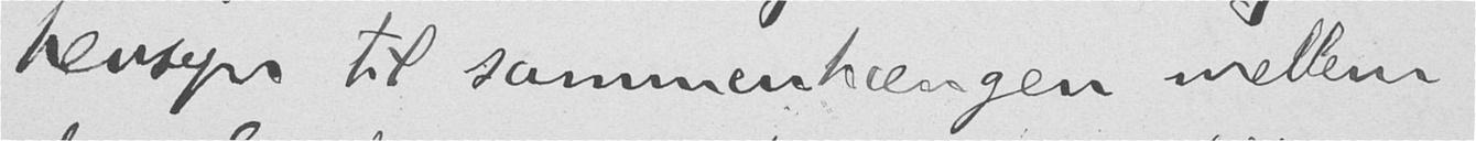hensyn til sammenhængen mellem
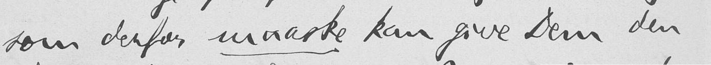som derfor maaske kan give Dem den
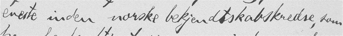eneste inden norske bekjendtskabskredse, som
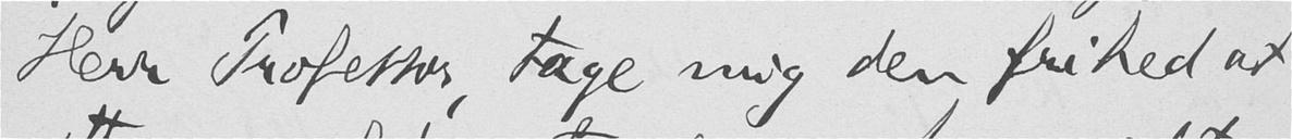Herr Professor, tage mig den frihed at
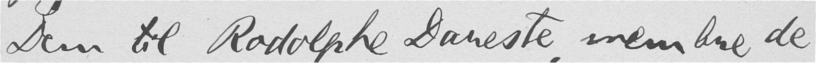Dem til Rodolphe Dareste, membre de
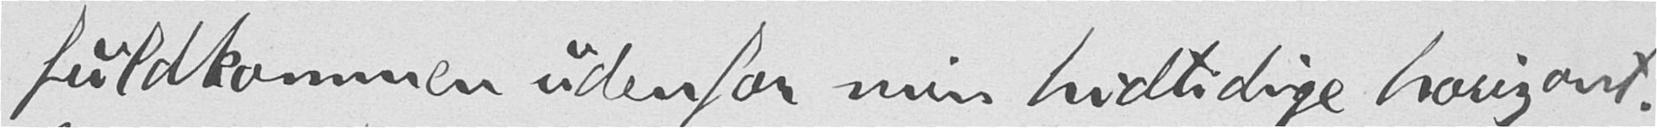fuldkommen udenfor min hidtidige horizont.
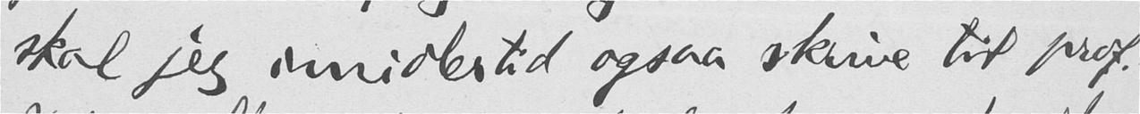skal jeg imidlertid ogsaa skrive til prof.
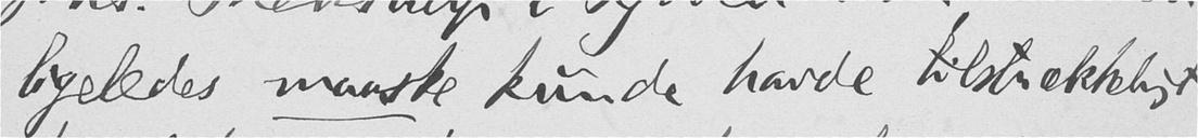ligeledes maaske kunde havde tilstrækkeligt
